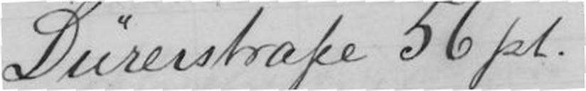Dürerstrasse 56 pl.
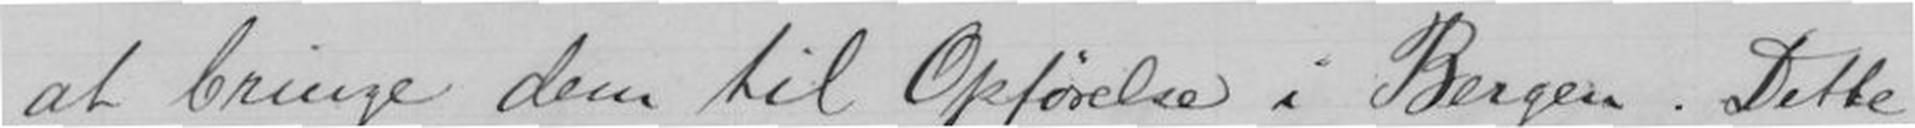at bringe dem til Opførelse i Bergen. Dette
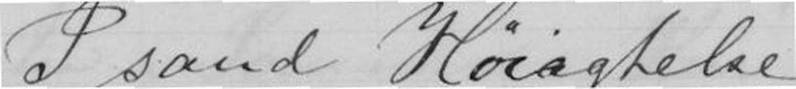I sand Høiagtelse
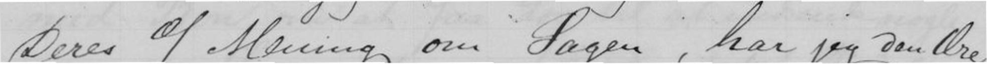Deres d/Mening om Sagen, har jeg den Ære
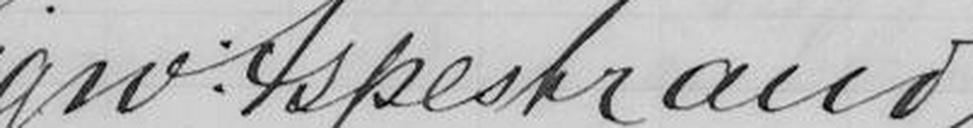Sigw. Aspestrand
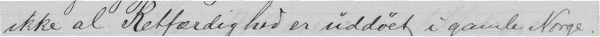ikke al Retfærdighed er uddøet i gamle Norge.
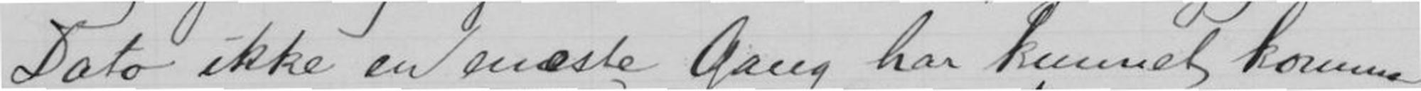Dato ikke en eneste Gang har kunnet komme
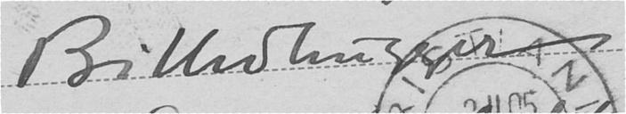Billedhugger
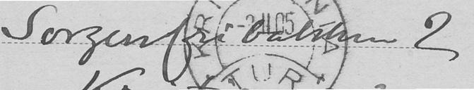Sorgenfribakken 2
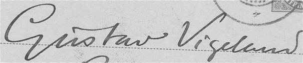Gustav Vigeland
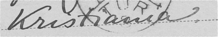Kristiania
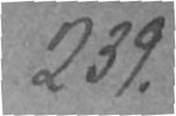239.
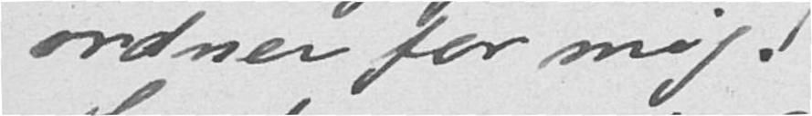ordner for mig.
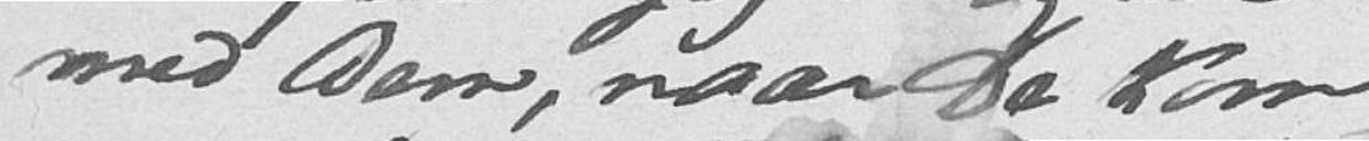med Dem, naar  De kom
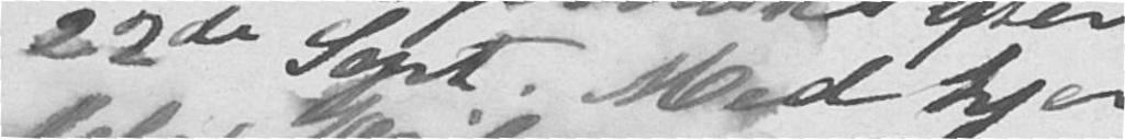22de Sept. Med hjer
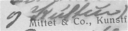og Kultur)
